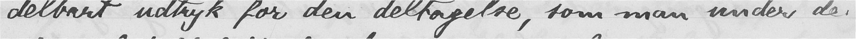delbart udtryk for den deltagelse, som man under de
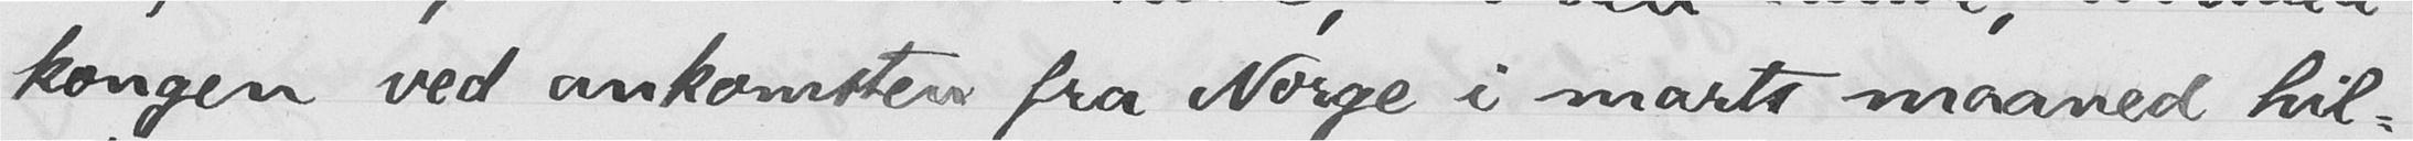kongen ved ankomsten fra Norge i marts maaned hil-
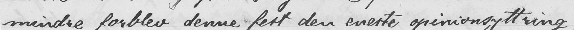mindre forblev denne fest den eneste opinionsyttring,
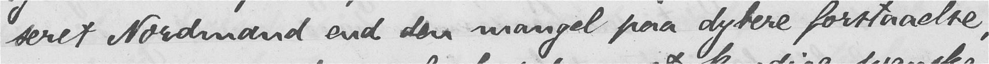seret Nordmand end den mangel paa dybere forstaaelse,
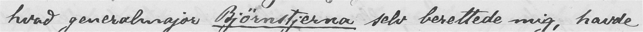hvad generalmajor Björnstjerna selv berettede mig, havde
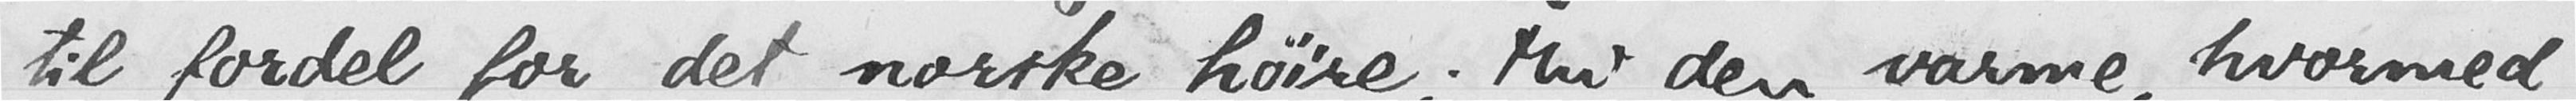til fordel for det norske høire; thi den varme, hvormed
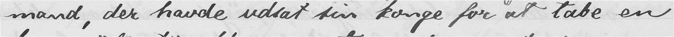mand, der havde udsat sin konge for at tabe en
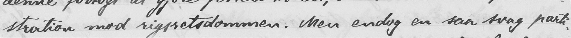stration mod rigsretsdommen. Men endog en saa svag parti-
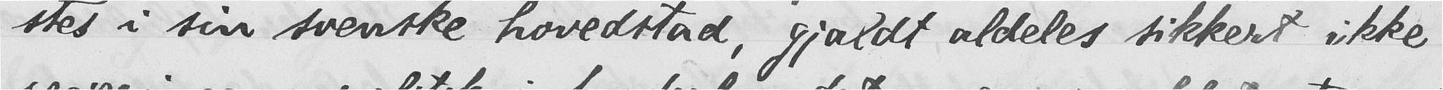stes i sin svenske hovedstad, gjaldt aldeles sikkert ikke
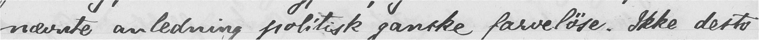nævnte anledning politisk ganske farveløse. Ikke desto
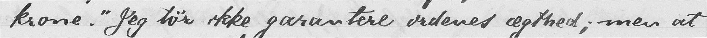krone." Jeg tør ikke garantere ordenes ægthed; men at
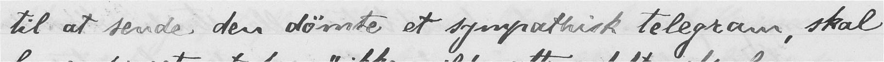til at sende den dømte et sympathisk telegram, skal
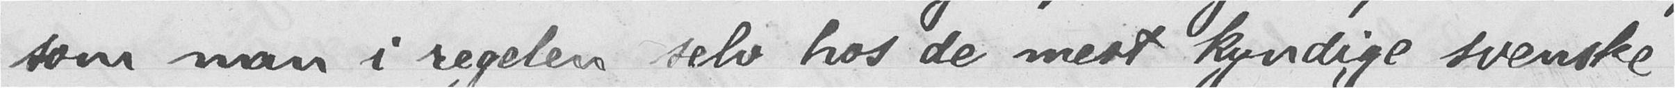som man i regelen selv hos de mest kyndige svenske
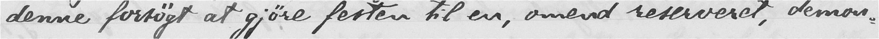denne forsøgt at gjøre festen til en, omend reserveret, demon-
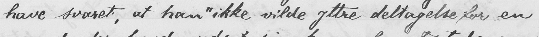have svaret, at han "ikke vilde yttre deltagelse for en
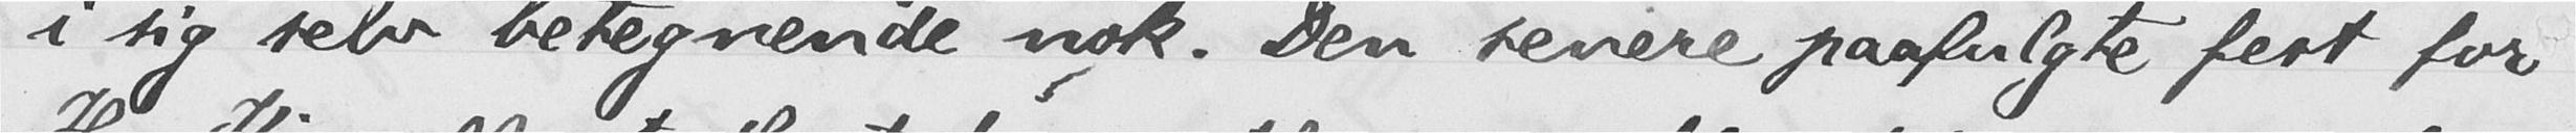i sig selv betegnende nok. Den senere paafulgte fest for
